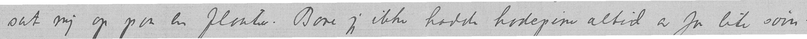sat mig op paa en flaate. Bare jeg ikke hadde hodepine altid av for lite søvn.
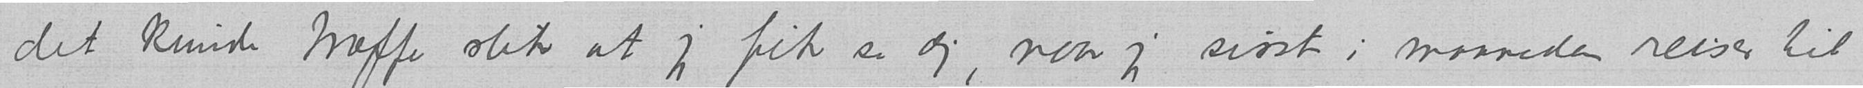det kunde træffe slik at jeg fik se dig, naar jeg sisst i maaneden reiser til
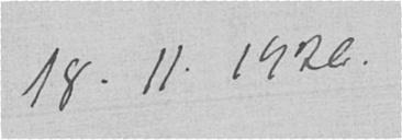18.11.1926.
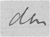din
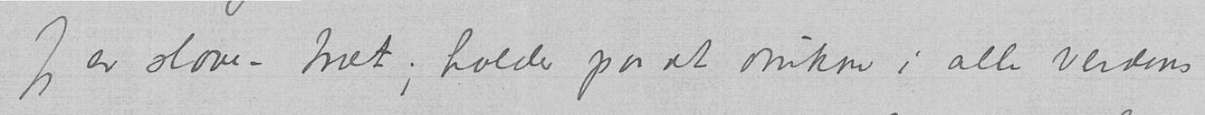Jeg er slave–træt; holder paa at drukne i alle verdens
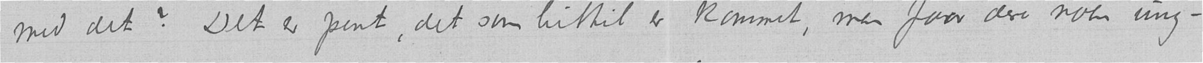med det? Det er pent, det som hittil er kommet, men faar dere noen ung-
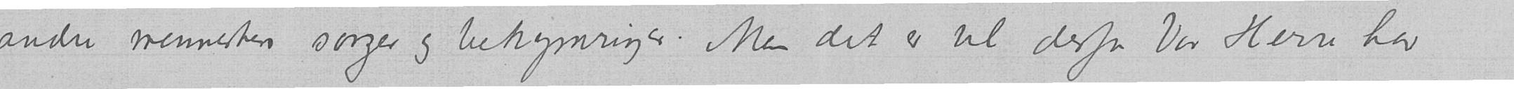andre menneskers sorger og bekymringer. Men det er vel derfor Vor Herre har
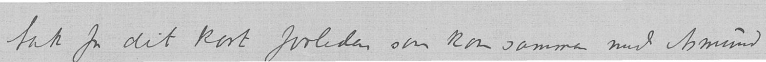tak for dit kort forleden som kom sammen med Asmund
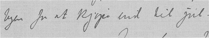byen for at kjøpe ind til jul.
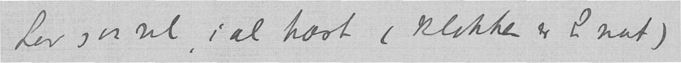Lev saa vel, i al hast (klokken er 2 nat)
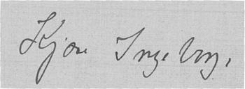Kjære Ingeborg,
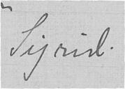Sigrid.
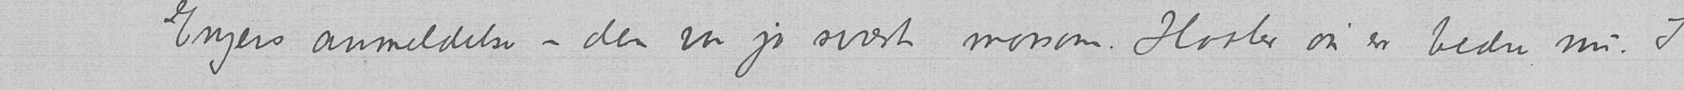Engers anmeldelse – den var jo svært morsom. Haaber du er bedre nu. Kanske
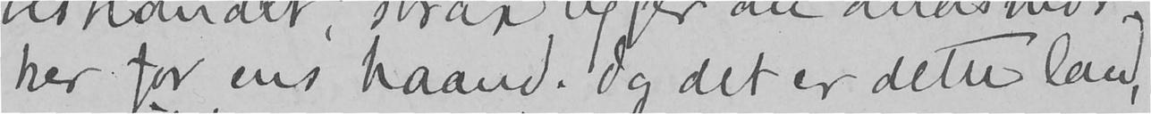ker for ens haand. Og det er dette land,
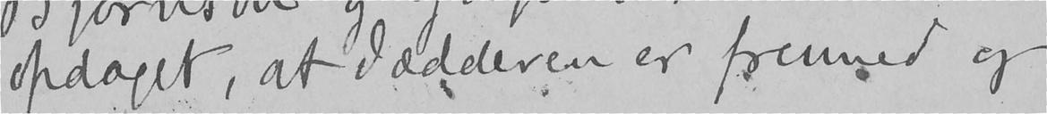opdaget, at Jædderen er fremmed og
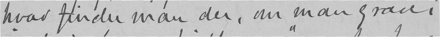hvad finder man der, om man graver i
